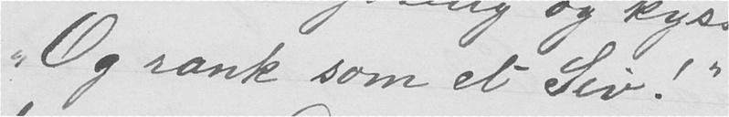"Og rank som et Siv!"
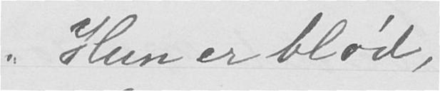"Hun er blød,
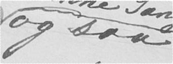og saa
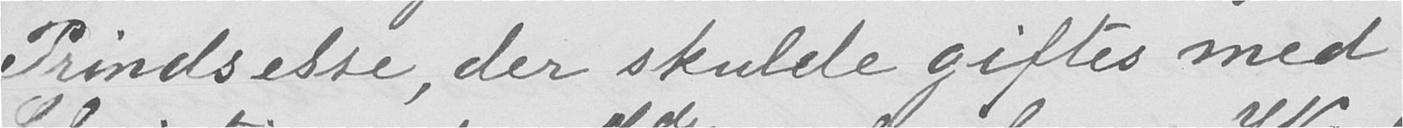Prindsesse, der skulde giftes med
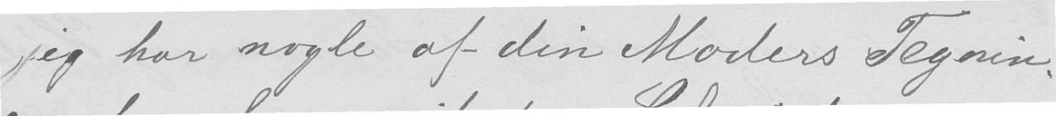jeg har nogle af din Moders Tegnin-
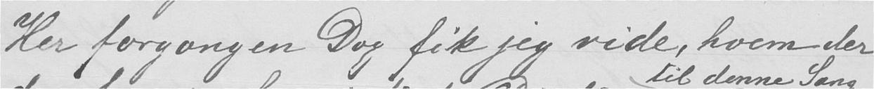Her forgangen Dag fik jeg vide, hvem der
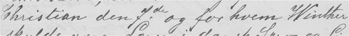Christian den 7de og for hvem Winther
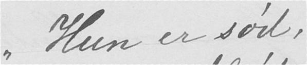"Hun er sød,
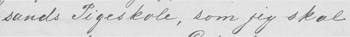sands Pigeskole, som jeg skal
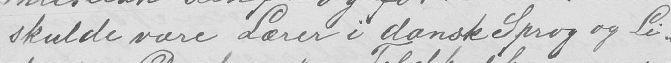skulde være Lærer i dansk Sprog og Li-
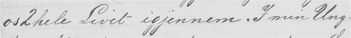os 2 hele Livet igjennem. I min Ung-
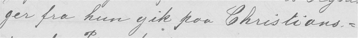ger fra hun gik paa Christians-
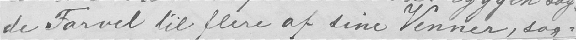de Farvel til flere af sine Venner, sag-
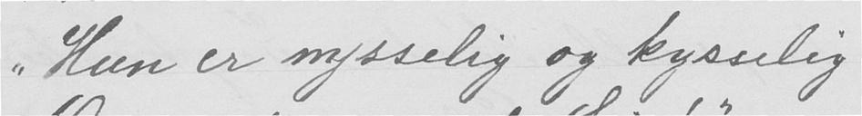"Hun er nysselig og kysselig
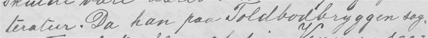teratur. Da han paa Toldbodbryggen sag-
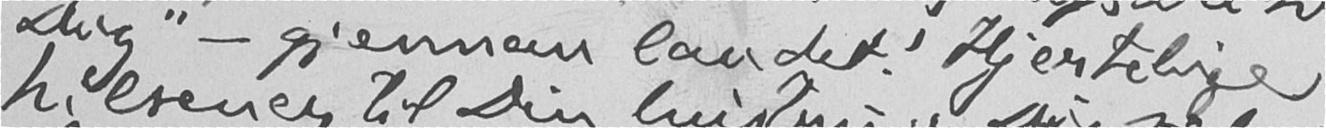Dig" – gjennem landet! Hjertelige
